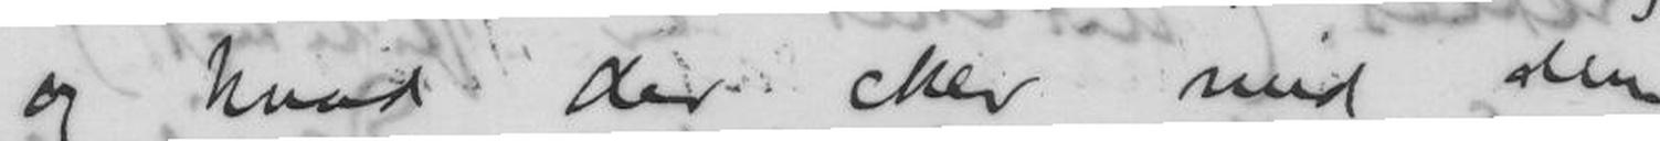og hvad der sker med dem,
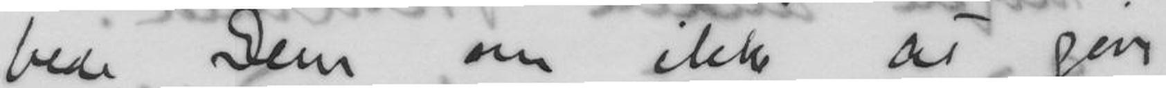bede Dem om ikke at give
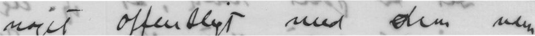noget offentligt med dem uden
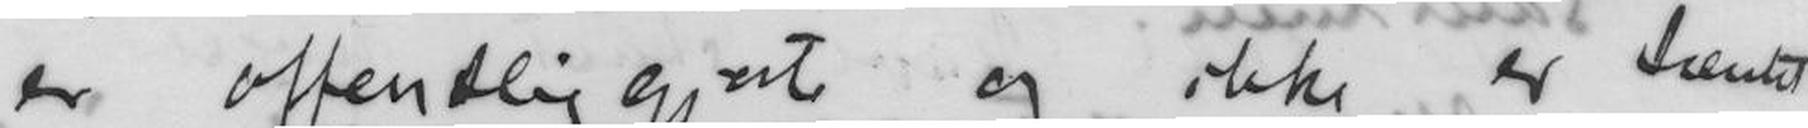er offentliggjort og ikke er tænkt
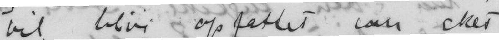vil blive opfattet som sket
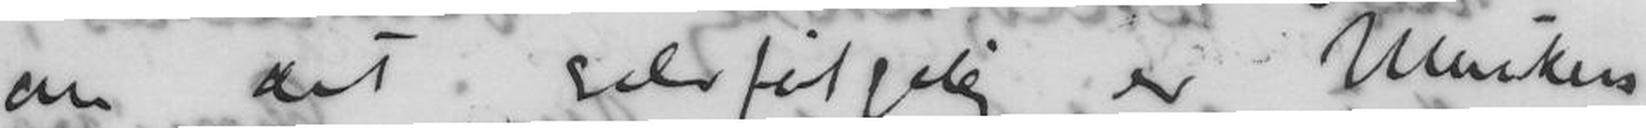om det selvfølgelig er Musiken
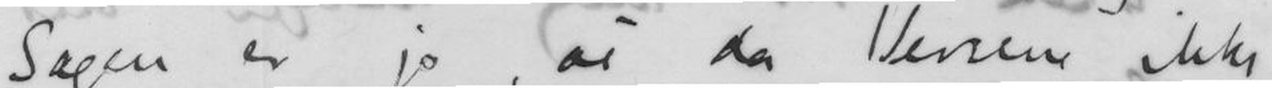Sagen er jo, at da Versene ikke
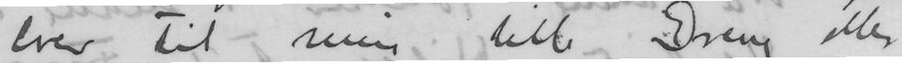til min lille Dreng eller
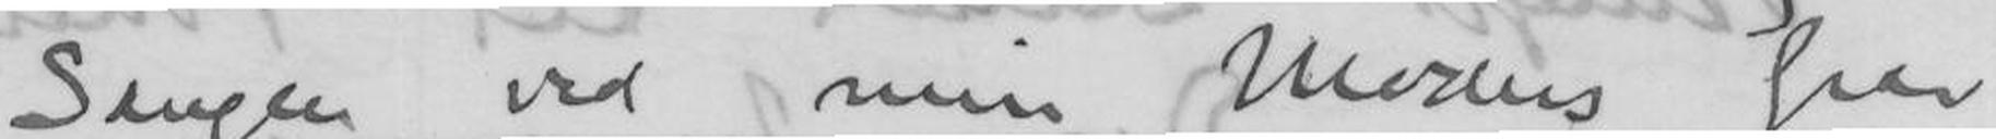sangen ved min Moders grav
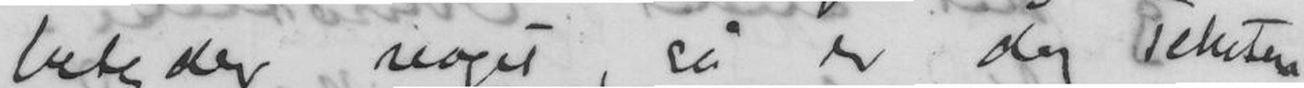betyder noget, så er dog Teksten
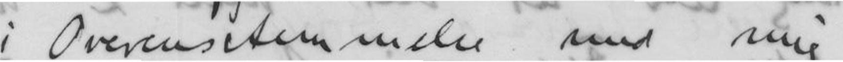i Overensstemmelse med mig
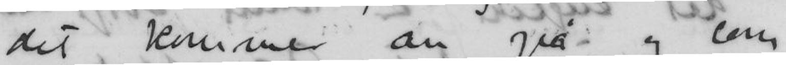det komme an på og som
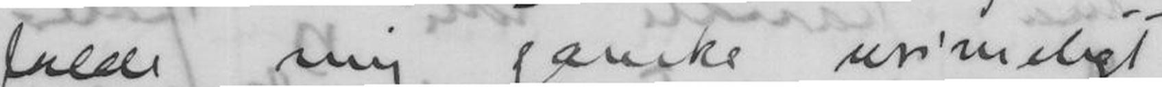falde mig ganske urimeligt
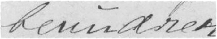beundrer
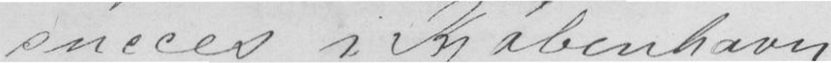Succes i Kjøbenhavn
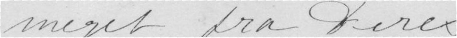meget fra Deres
